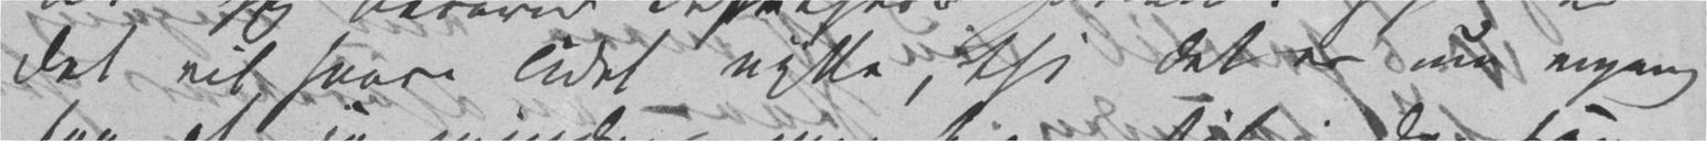det vil saare lidet nytte, thi det er nu engang
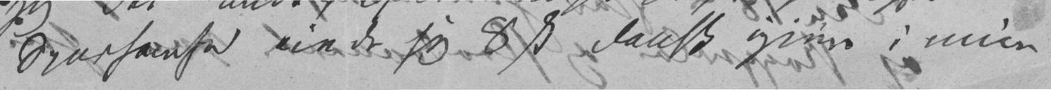Sparsomhed eiede jeg 8 [Skilling] dansk igjen i min
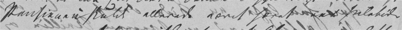Pensionen skulde allerede være hævet ved Juletider
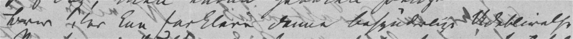Brev der kan forklare denne besynderlige Udeblivelse
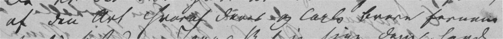af den Art hvoraf Deres og Carls Breve fornem
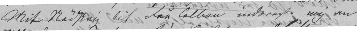Mit Nødskrig til Fru Colban indbragte mig en
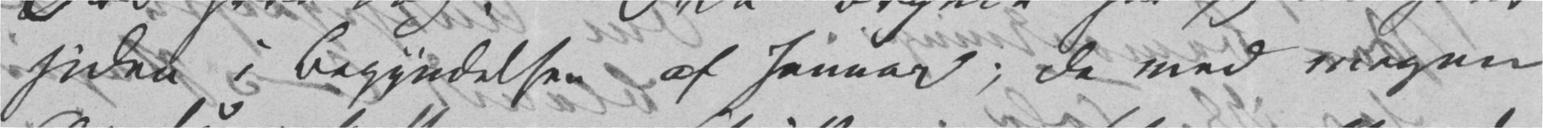siden i Begyndelsen af Januar; de med megen
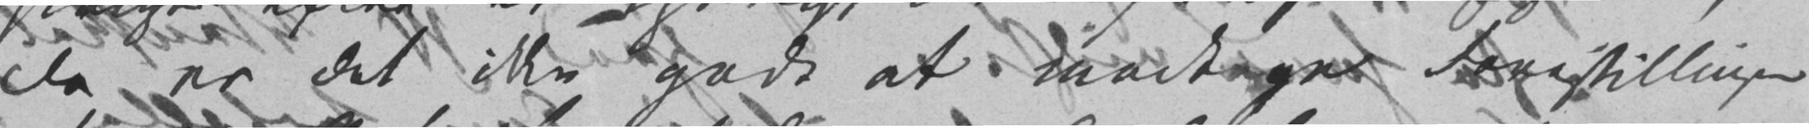da er det ikke godt at modtage Forestillinger
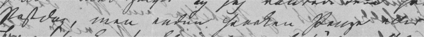Postdag, men endnu hverken Penge eller
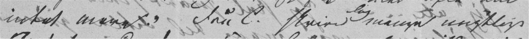«intet mere.» Fru C. skriver dog mange unyttige
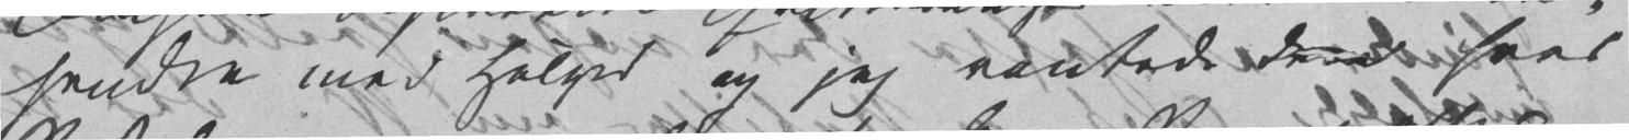sendte med Holger og jeg ventede dem hver
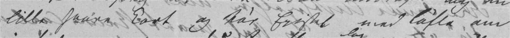lille saare kort og tør Epistel med Løfte om
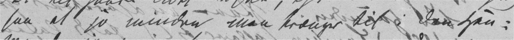saa at jo mindre man trænger til i den Hen-
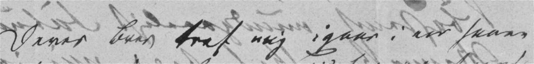Deres Brev traf mig igaar i en saare
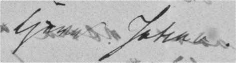Kjere Johan.
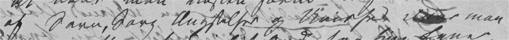af Savn, Sorg Ængstelser og Uvished naar man
